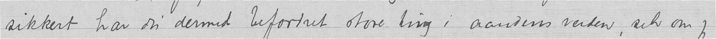sikkert har du dermed befordret store ting i aandens verden, selv om jeg
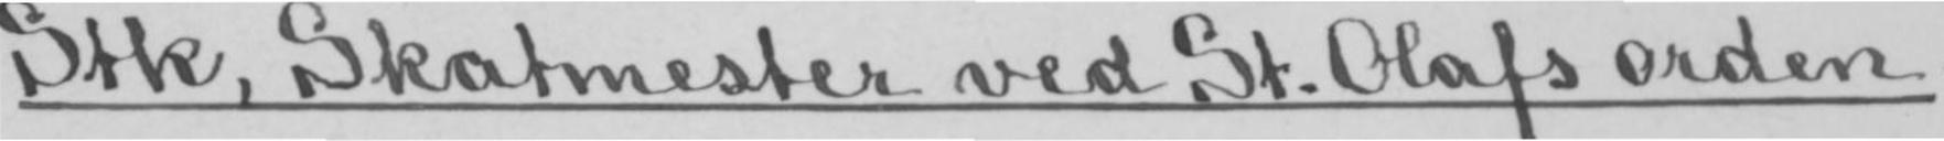Stk, Skatmester ved St. Olafs orden.
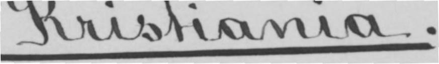Kristiania.
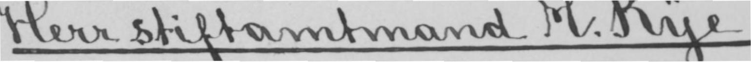Herr stiftamtmand M. Rye
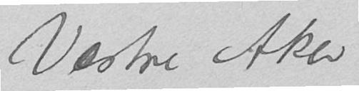Vestre Aker
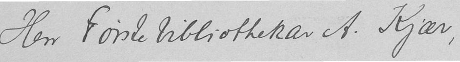Herr Førstebibliothekar A. Kjær,
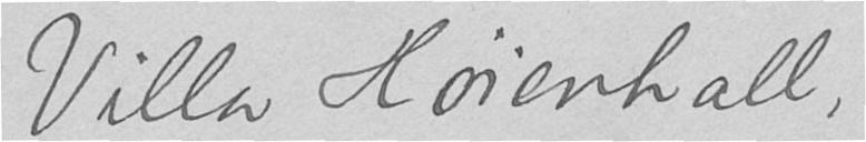Villa Høienhall,
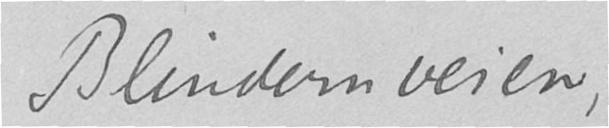Blindernveien,
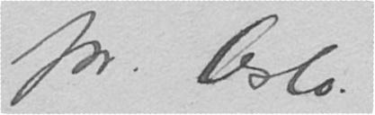pr. Oslo.
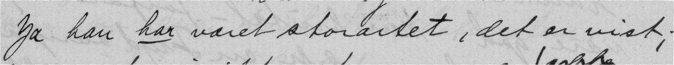Ja han har været storartet, det er vist;
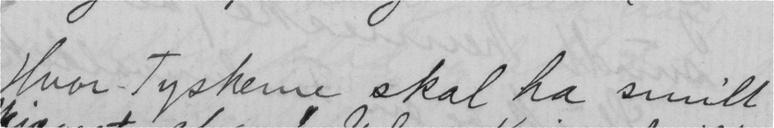Hvor Tyskerne skal ha smilt
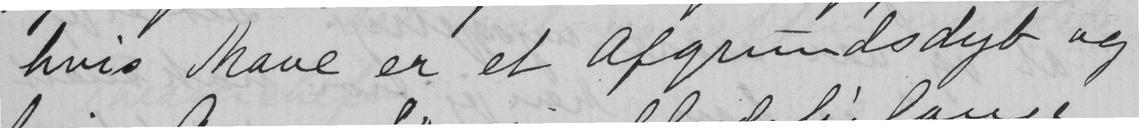hvis Mave er et Afgrundsdyb og
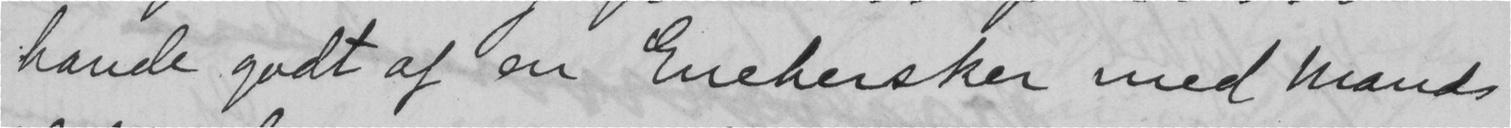havde godt af en Enehersker med mands
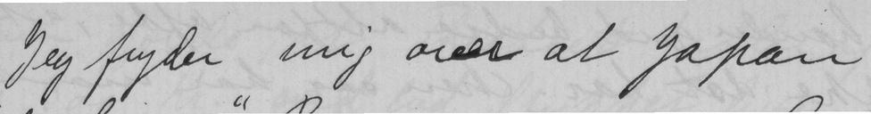Jeg fryder mig over at Japan
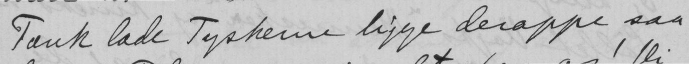Tænk lade Tyskerne ligge deroppe saa
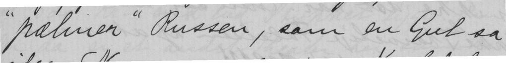"pælmer" Russen, som en Gut sa
